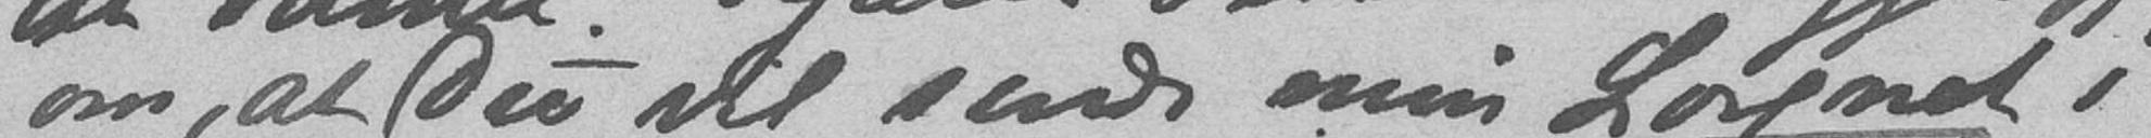om, at Du vil sende min  i
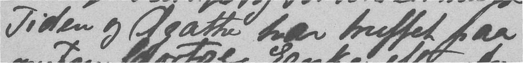Tiden og Agathe har hyfet paa
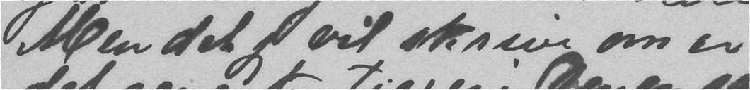Men det jeg vil skrive om er
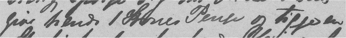give hende 1 Krones Penge og tigge en
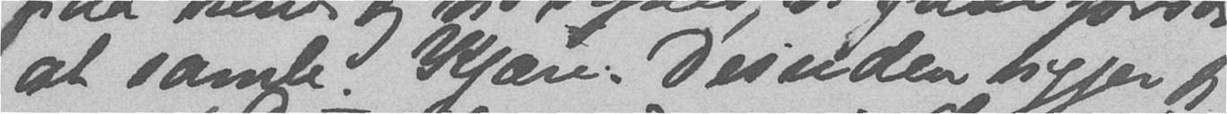at samle. Kjære. Desuden tigger jeg
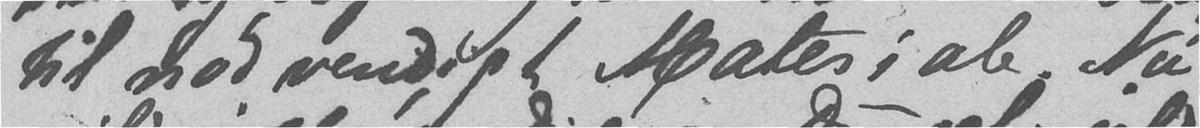til nødvendigt Materiale. Nu
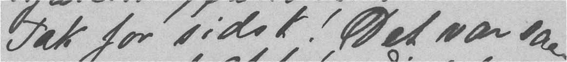Tak for sidst! Det var saa
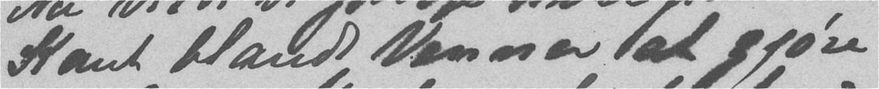Kant blandt Venner at gjøre
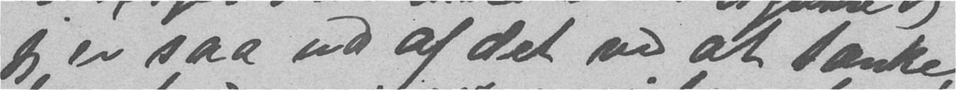jeg er saa ud af det ved at tænke
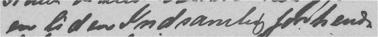en liden Indsamlig for hende.
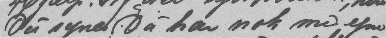Du synes Du har nok med egne
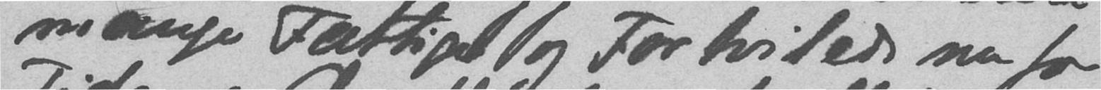mange Fattige og Fortvilede nu for
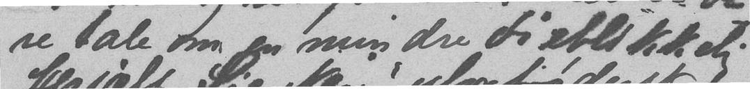re tale om en mindre Øieblikkelig
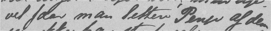vel faar man lettere Penge a den
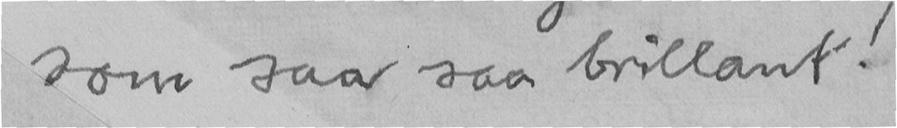som saa saa brillant!
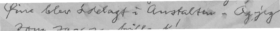Øine blev ødelagt i Anstalten. Og jeg
